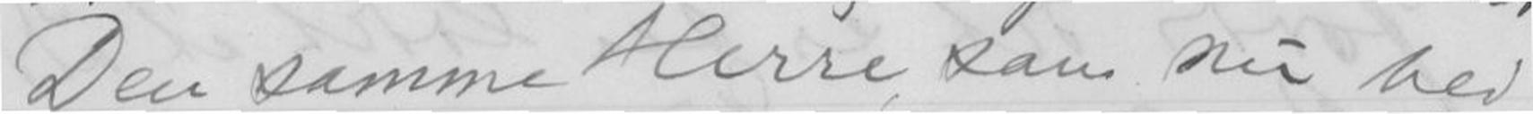Den samme Herre som nu ved
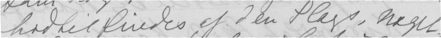hidtil findes af den Slags, noget
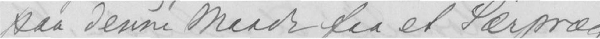paa denne Maade faa et Særpræg
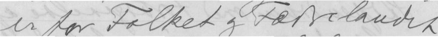er for Folket og Fædrelandet.
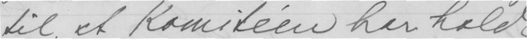til, at Komitéen har holdt
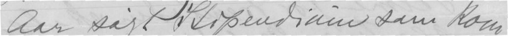Aar søgt Stipendium som "Kom-
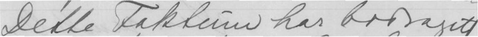Dette Faktum har bridraget
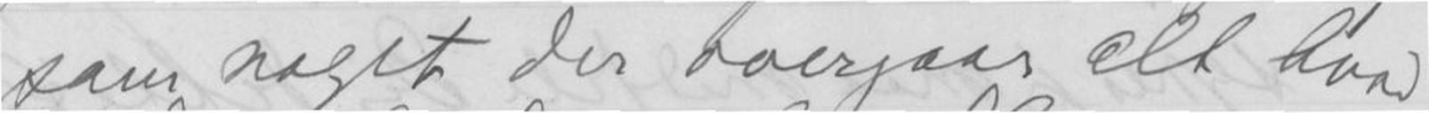som noget der overgaar alt hvad
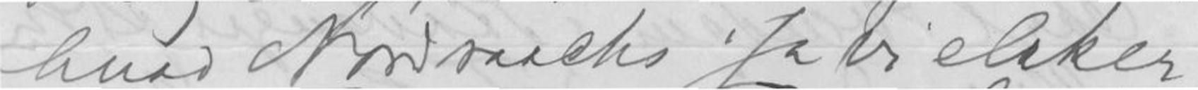hvad Nordraachs "Ja vi elsker"
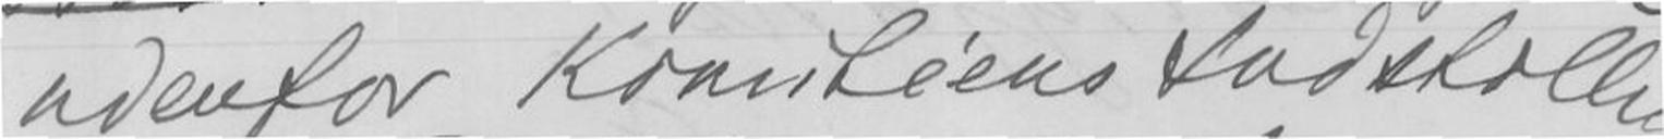udenfor Komitéens Indstilling.
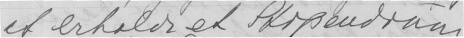at erholde et Stipendium
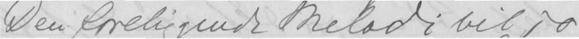Den foreligende Melodi vil jo
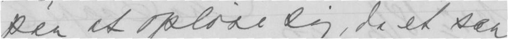paa at opløse sig, da et saa
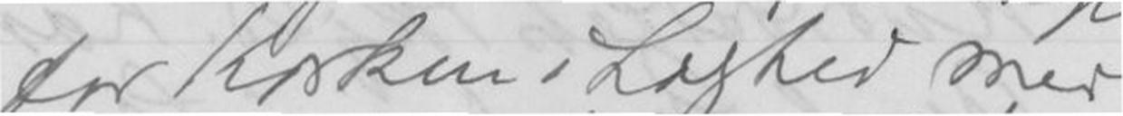for Kirken i Lighed med
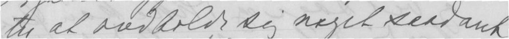til at indholde sig noget saadant.
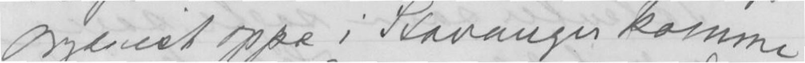Organist oppe i Stavanger komme
